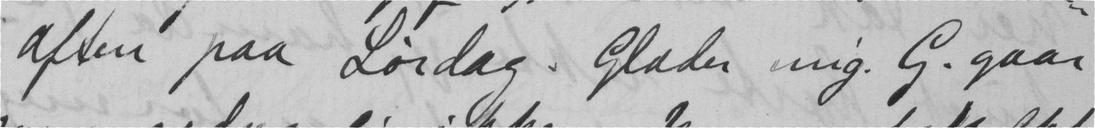aften paa Lørdag. Glæder mig. G. gaar
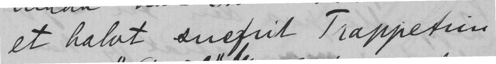et halvt snefrit Trappetrin
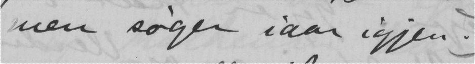men søger iaar igjen.
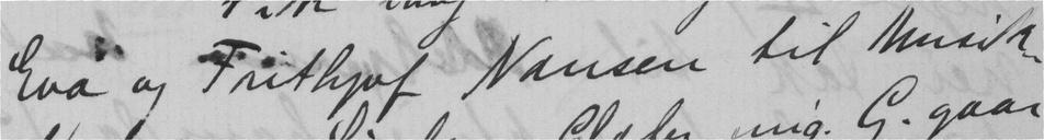Eva og Frithjof Nansen til Musik-
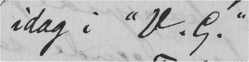idag i "V. G."
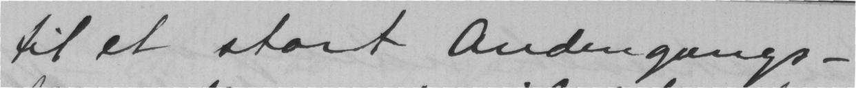til et stort Andengangs-
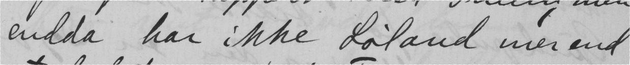endda har ikke Løland mer end
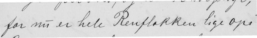for nu er hele Renflokken lige opå
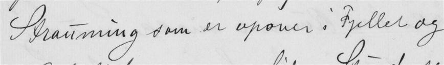Strauming som er opover i Fjellet og
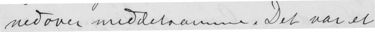nedover meddetsamme. Det var et
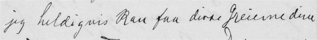jeg heldigvis kan faa disse Greiene dine
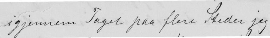igjennem Taget paa flere Steder jeg
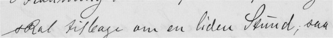skal tilbage om en liden Stund, saa
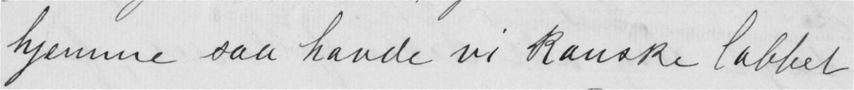hjemme saa havde vi kanske labbet
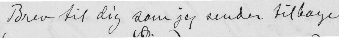Brev til dig som jeg sender tilbage
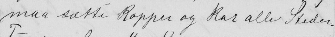maa sætte Kopper og Kar alle Steder -
